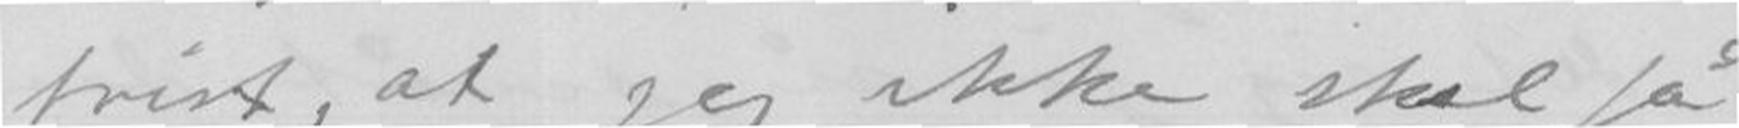trist, at jeg ikke skal få
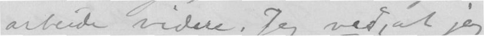arbeide videre. Jeg ved, at jeg
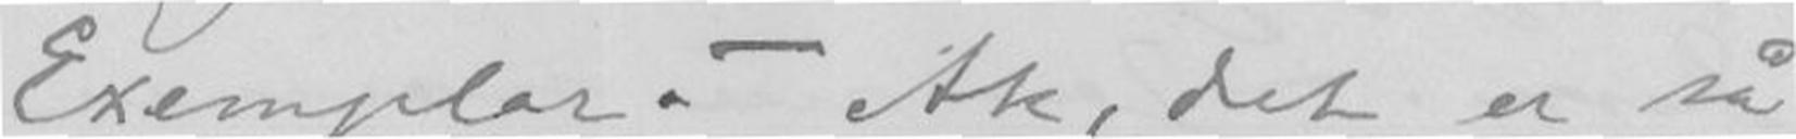Exemplar. - Ak, det er så
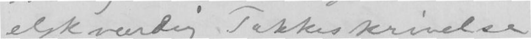elskværdig Takkeskrivelse
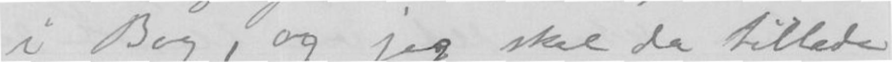i Bog, og jeg skal da tillade
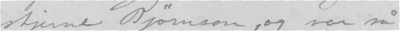stjerne Bjørnson, og var så
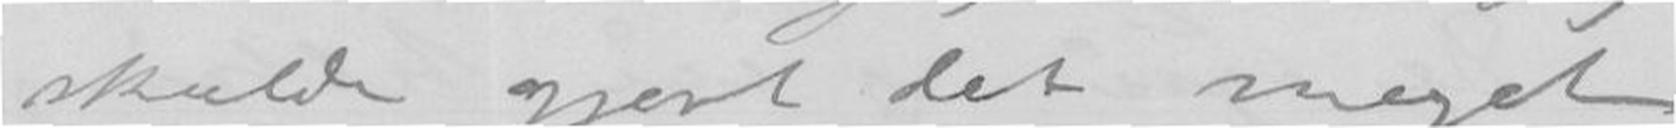skulde gjort det meget
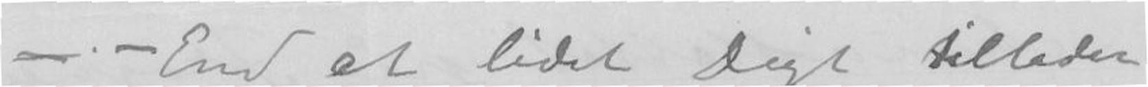- - End et lidet Digt tillader
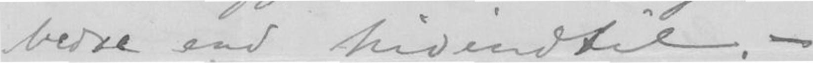bedre end hidindtil.
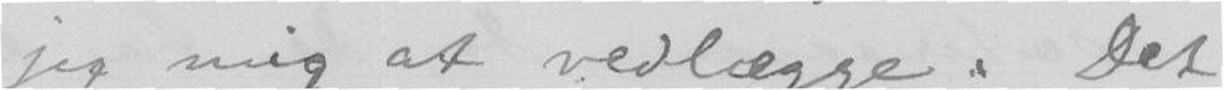jeg mig at vedlegge. Det
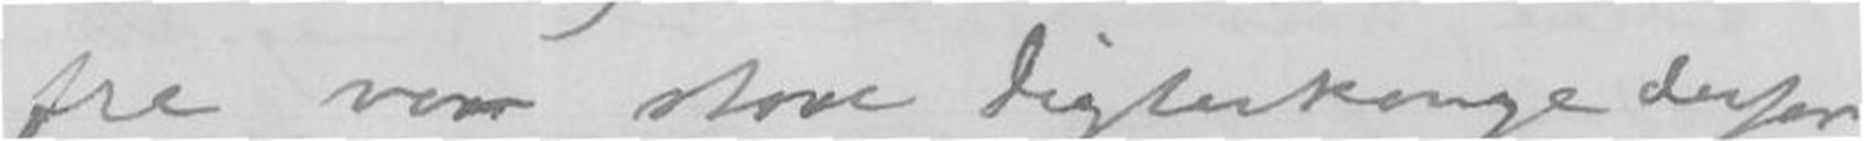fra vor store digterkonge derfor,
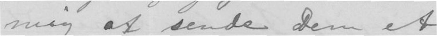mig at sende Dem et
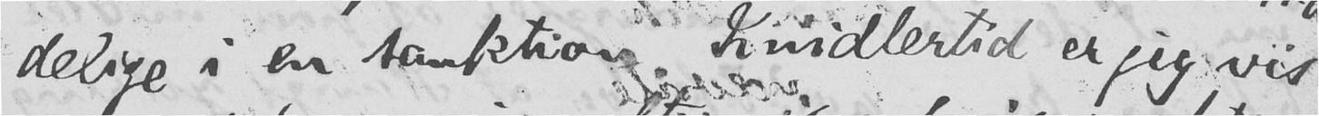delige i en sanktion. Imidlertid er jeg vis
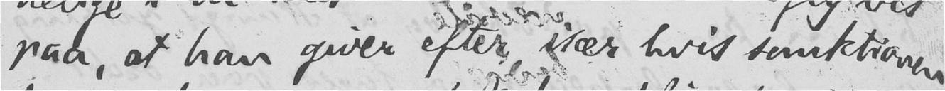paa, at han giver efter, især hvis sanktionen
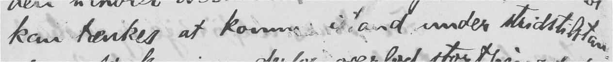kan tænkes at komme istand under stridstilstan-
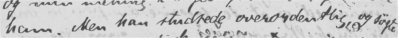ham. Men han studsede overordentlig, og søgte
